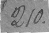210.
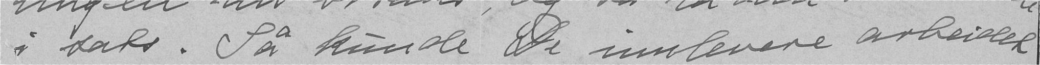i sats. Så kunde De innlevere arbeidet
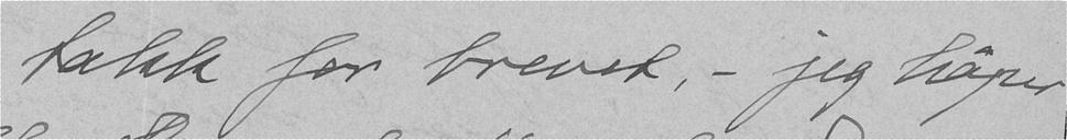takk for brevet, - jeg håper
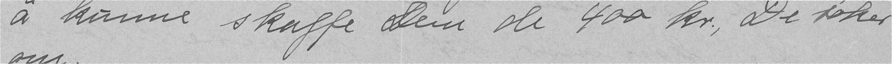å kunne skaffe Dem de 400 kr., De søker
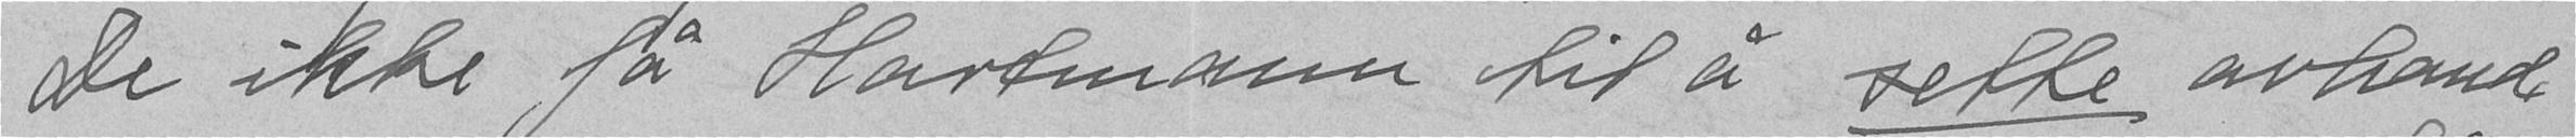De ikke få Hartmann til å sette avhand-
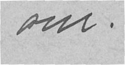om.
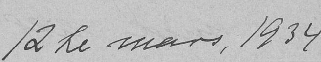12te mars, 1934
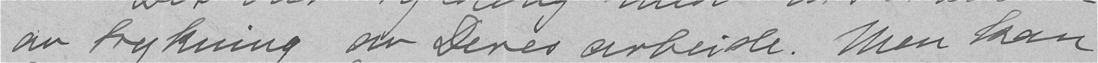av trykning av Deres arbeide. Men kan
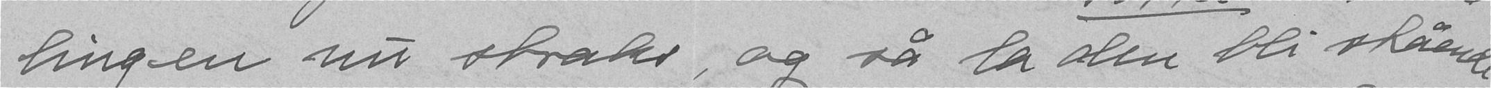lingen nu straks, og så la den bli stående
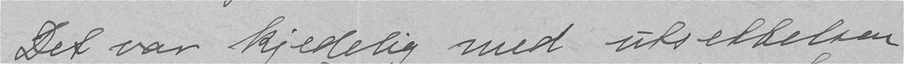Det var kjedelig med udsettelsen
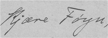Kjære Føyn,
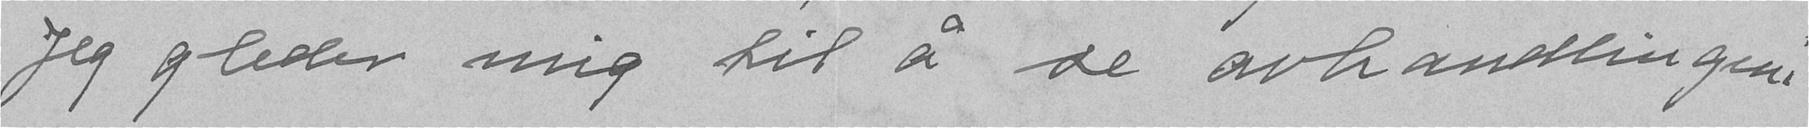Jeg gleder mig til å se avhandlingen!
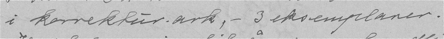i korrektur-ark, - 3 eksemplarer.
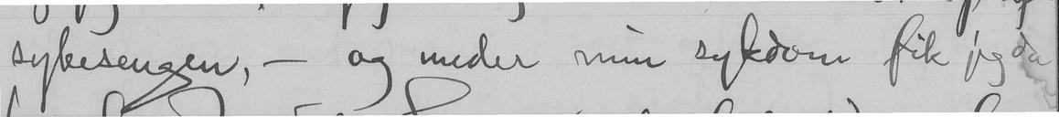sykesingen, - og under min sykdom fik jeg da
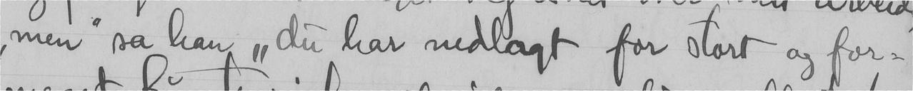"men" sa han "du har nedlagt for stort og for-
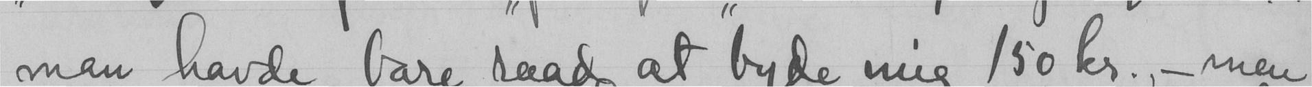man havde bare raad at byde mig 150kr., - men
